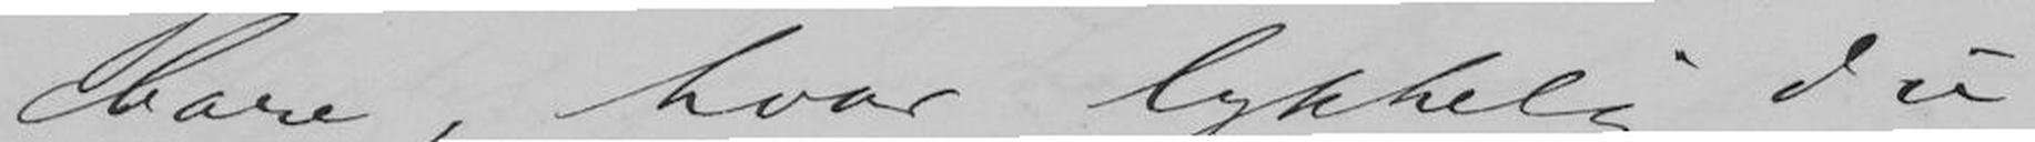bare, hvor lykkelig Du
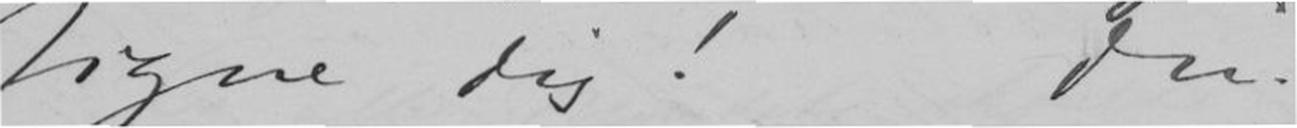signe dig!Din
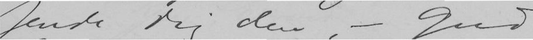sende Dig den, - Gud
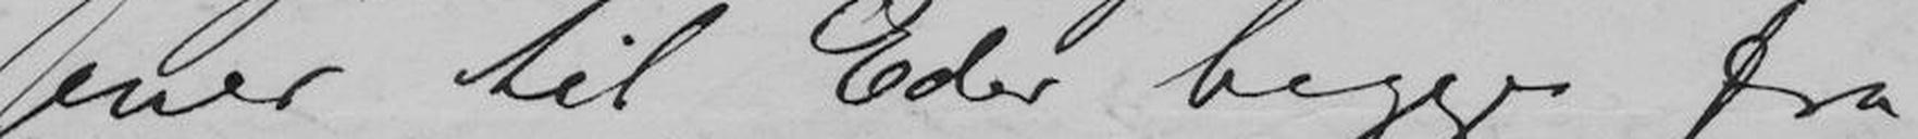sener til Eder begge fra
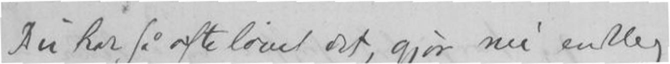Du har så ofte lovet det, gjør nu endelig
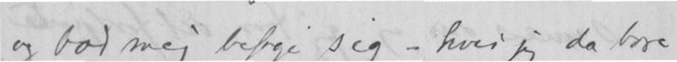og bad mig besøge sig - hvis jeg da bare
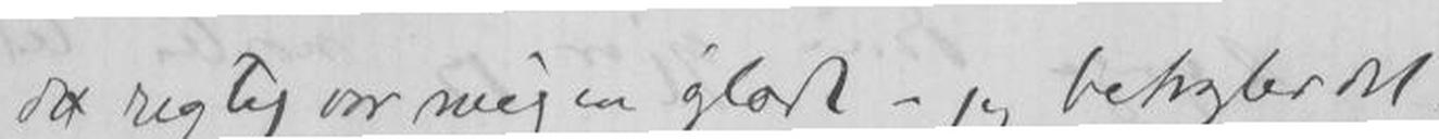det rigtig var mig en glæde - jeg betragter det
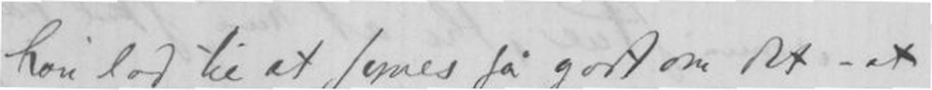han lod til at synes så godt om det - at
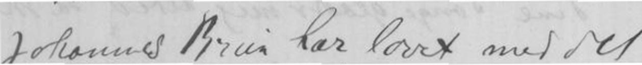Johannes Brun har lovet med det
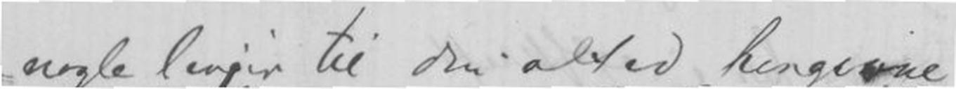nogle linjer til din altid hengivne
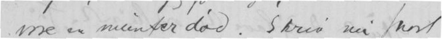være en munter død. Skriv nu snart
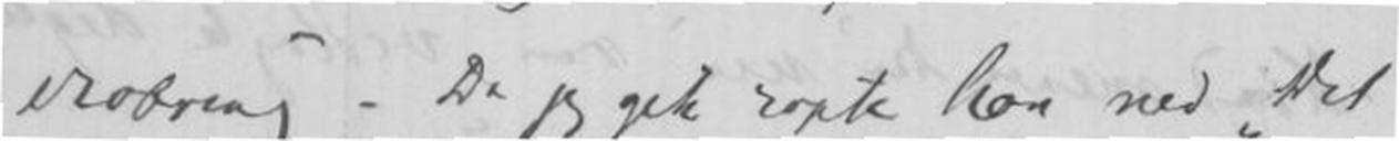erobring - Da jeg gik ropte han ned Det
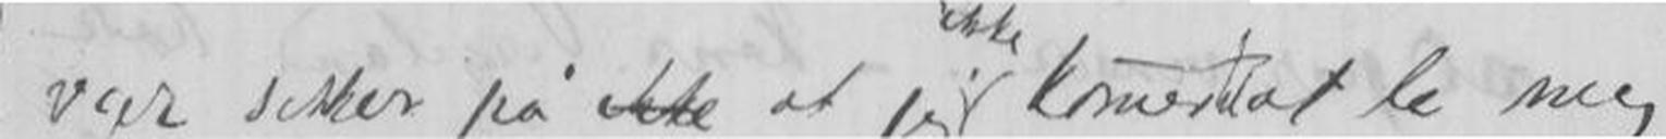var sikker på ikke at jeg komer til at le mig
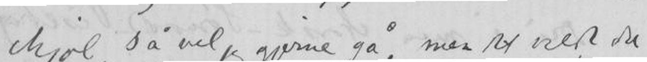ihjæl, så vil jeg gjerne gå, men det vilde da
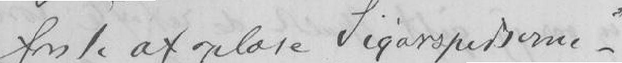første at oplæse "Sigarspedserne"
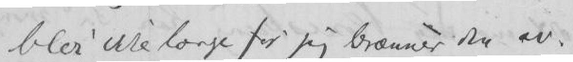blir ikke længe før jeg brænner den av!
